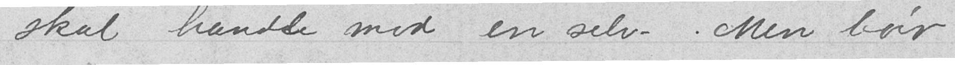skal handle mod en selv. Men bør
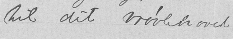til dit vrøvlehoved
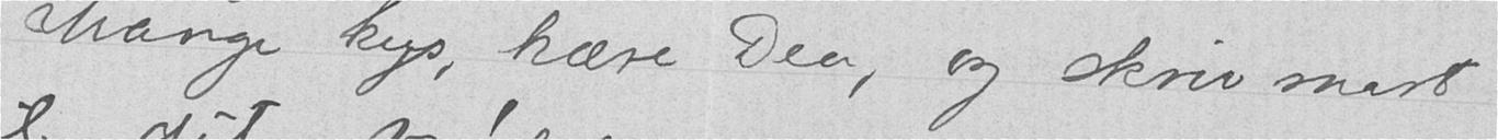Mange kys, kære Dea, og skriv snart
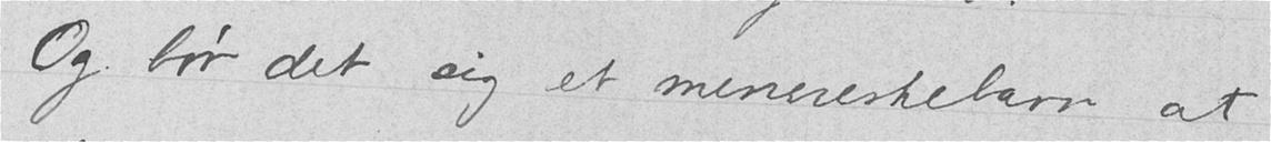Og bør det sig et menneskebarn at
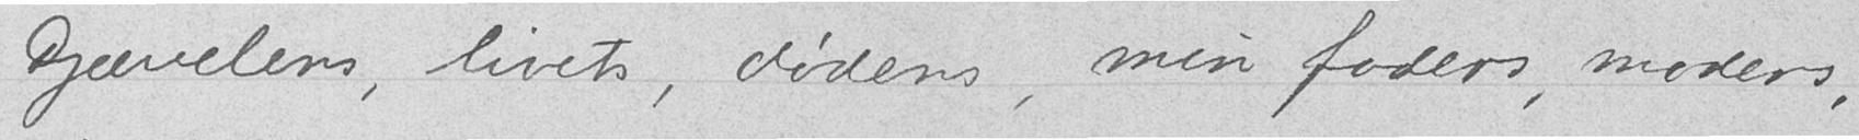Djævelens, livets, dødens, min faders, moders,
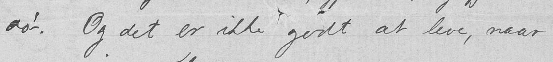dø. Og det er ikke godt at leve, naar
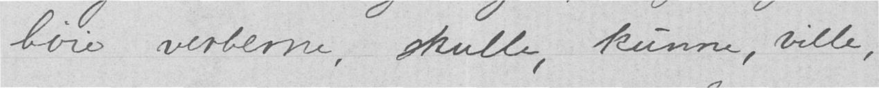bøie verberne, skulle, kunne, ville,
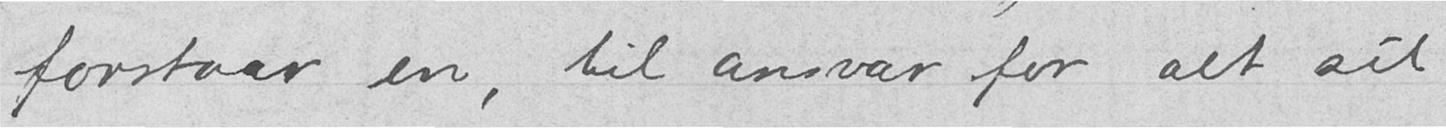forstaar en, til ansvar for alt sit
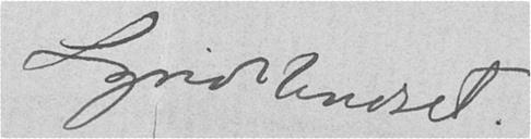Sigrid Undset.
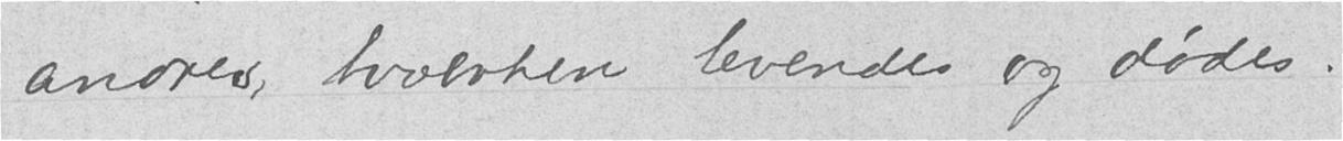andres, hverken levendes eller dødes.
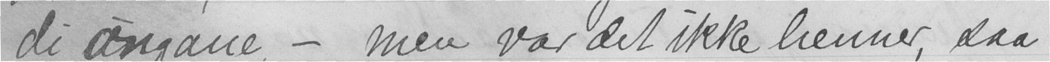de ungane, - men var det ikke henner, saa
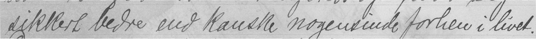sikkert bedre end kanske nogensinde forhen i livet.
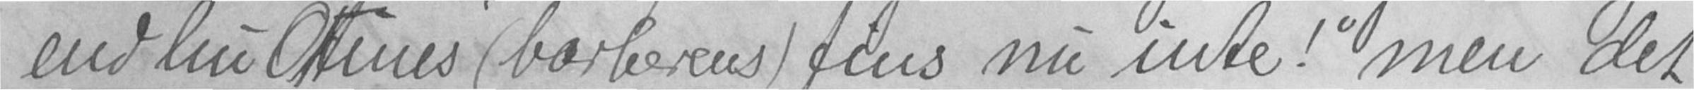end hu Ottines (barberens) fins nu inte!" men det
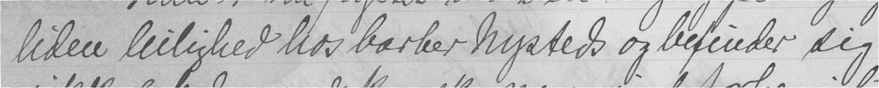liden leilighed hos barber Nysteds og befinder sig
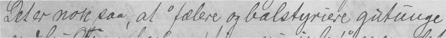Det er nok saa, at "fælere og balstyriere gutunge
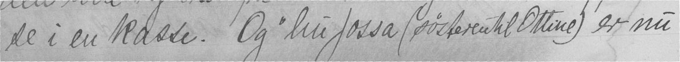se i en kasse. Og "hu Jossa (søsteren til Ottine) er nu
